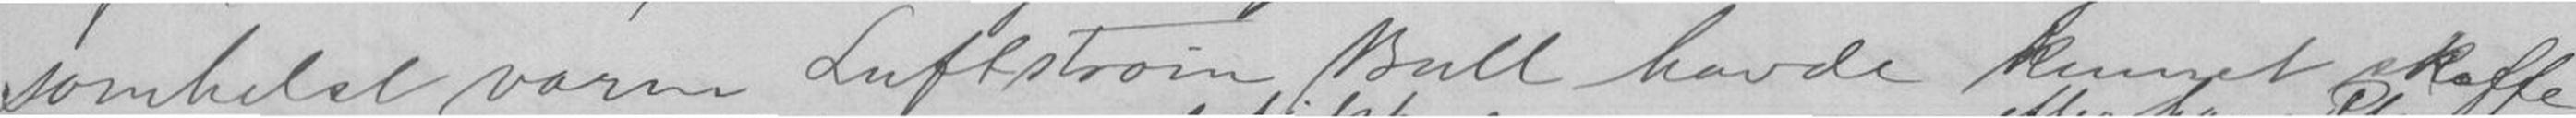somhelst varm Luftstrøm Bull havde kunnet skaffe
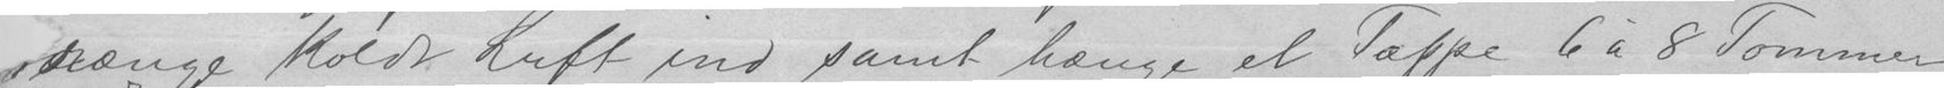trænge kold Luft ind, samt hænge et Tæppe 6 à 8 Tommer
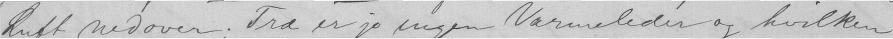Luft nedover; Træ er jo ingen Varmeleder og hvilken
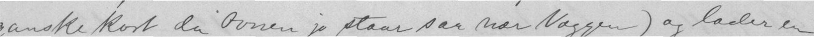ganske kort da Ovnen jo staar saa nær Væggen) og lader en
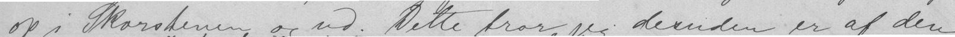op i Skorstenen og ud. Dette tror jeg desuden er af den
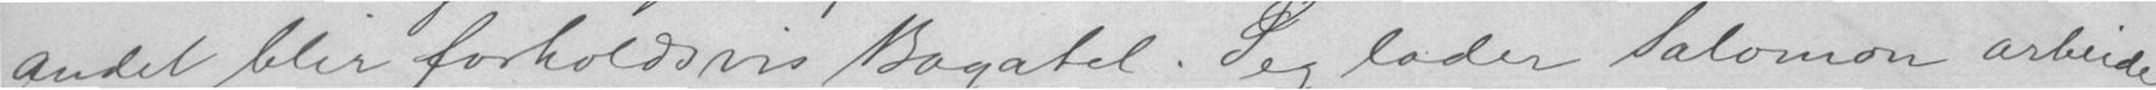andet blir forholdsvis Bagatel. Jeg lader Salomon arbeide
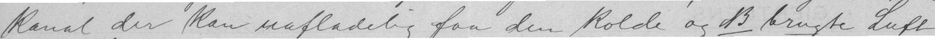kanal der kan uafladelig faa den kolde og NB brugte Luft
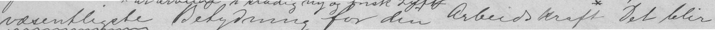væsentligste Betydning for din Arbeidskraft* Det blir
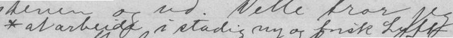* at arbeide i stadig ny og frisk Luft
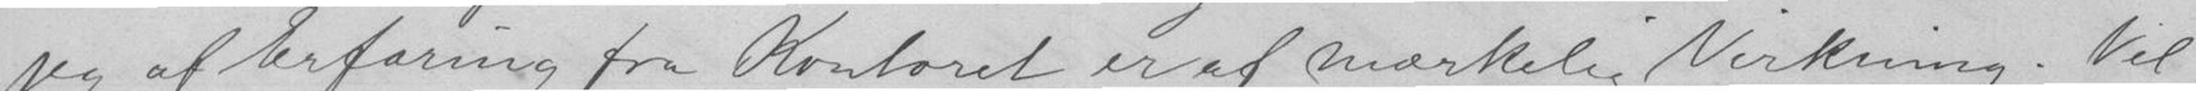jeg af Erfaring fra kontoret er af mærkelig Virkning. Vil
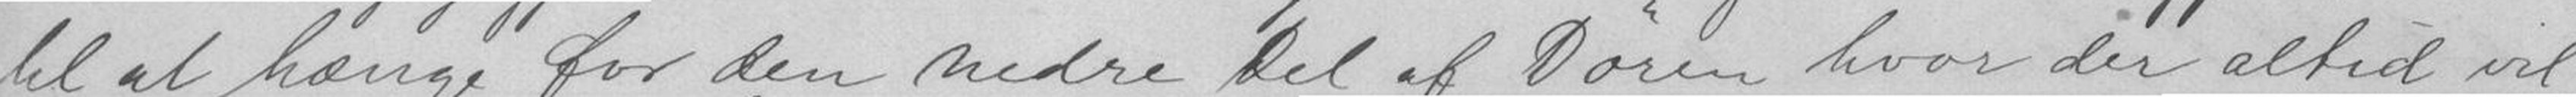til at hænge for den nedre Del af Døren hvor der altid vil
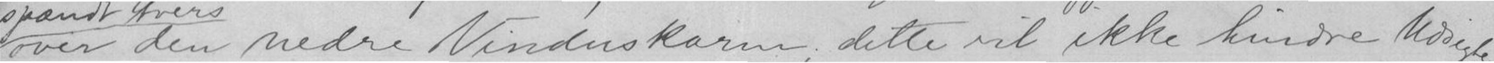over den nedre Vinduskarm; dette vil ikke hindre Udsigten
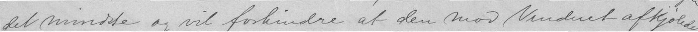det mindste og vil forhindre at den mod Vinduet afkjølede
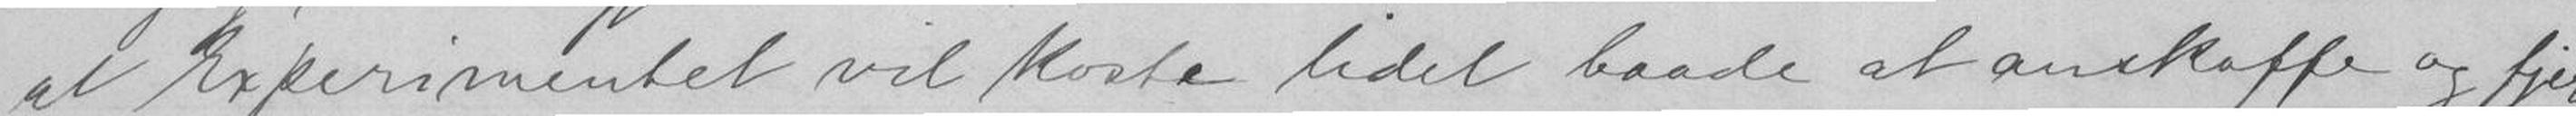at Experimentet vil koste lidet baade at anskaffe og fjerne
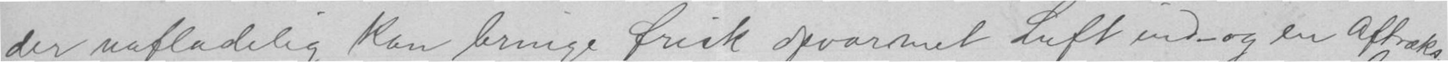der uafladelig kan bringe frisk opvarmet Luft ind - og en Aftræks
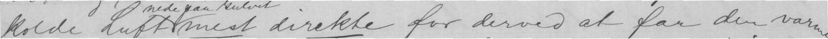kolde Luft mest direkte for derved at faa den varme
